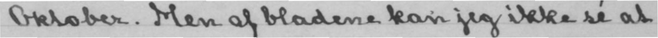Oktober. Men af bladene kan jeg ikke sé at
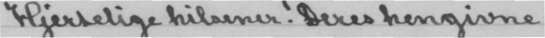Hjertelige hilsener! Deres hengivne
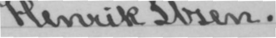Henrik Ibsen.
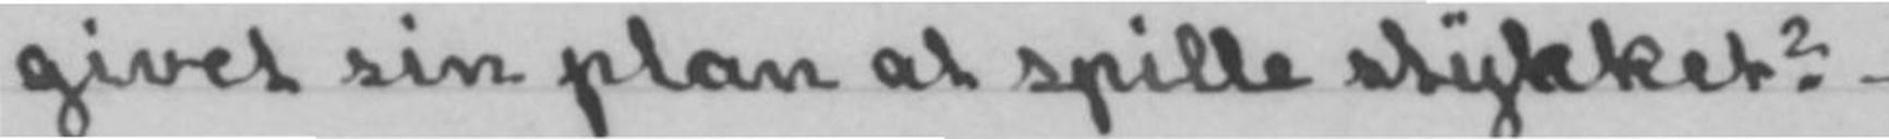givet sin plan at spille stykket?-
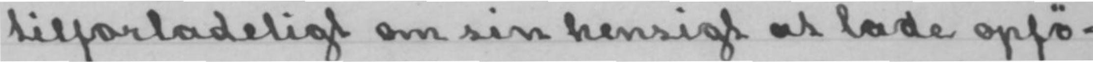tilforladeligt om sin hensigt at lade opfø-
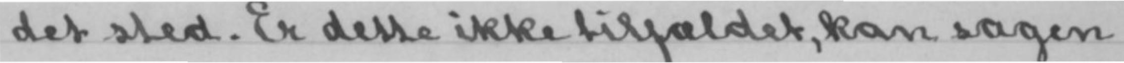det sted. Er dette ikke tilfældet, kan sagen
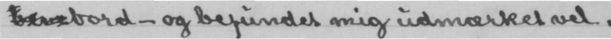benbord- og befundet mig udmærket vel.
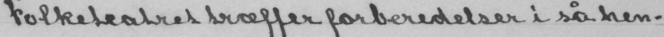Folketeatret træffer forberedelser i så hen-
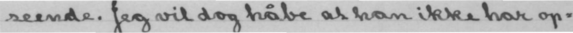seende. Jeg vil dog håbe at han ikke har op-
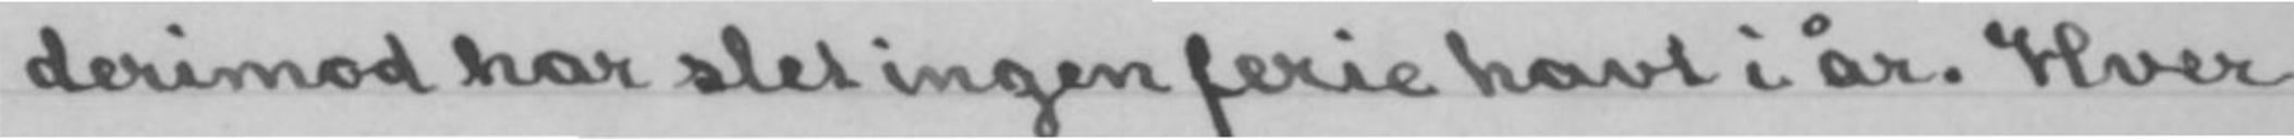derimod har slet ingen ferie havt i år. Hver
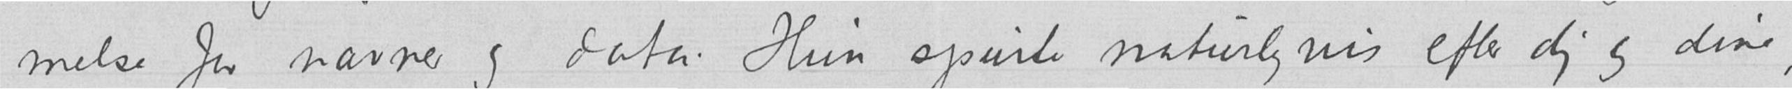melse for navner og data. Hun spurte naturligvis efter dig og dine,
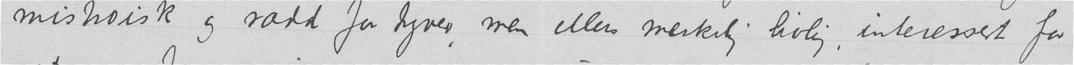mistroisk og rædd for tyver, men ellers merkelig livlig, interessert for
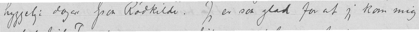hyggelige dager praa Rødkilde. Jeg er saa glad for at jeg kom mig
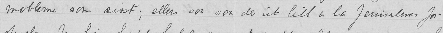møblerne som sisst; ellers saa saa der ut litt a la Jerusalems for-
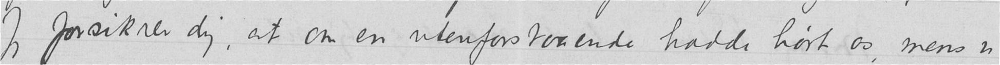Jeg forsikrer dig, at om en utenforstaaende hadde hørt os, mens vi
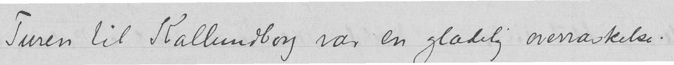Turen til Kallundborg var en glædelig overraskelse.
